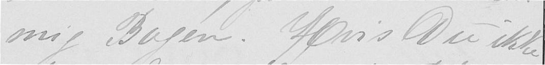mig Bogen. Hvis Du ikke
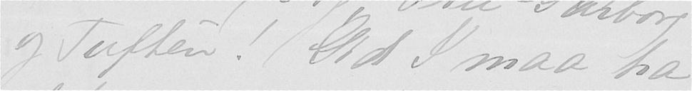og Tuften! Gid I maa ha
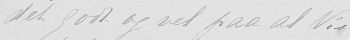det godt og vel paa al Vis
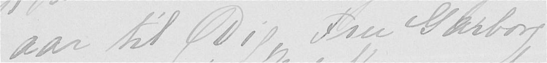aar til Dig, Fru Garborg
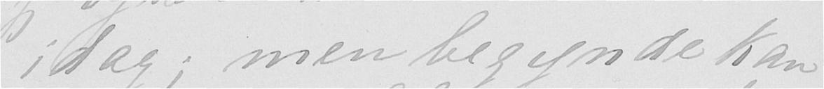i dag; men begynde kan
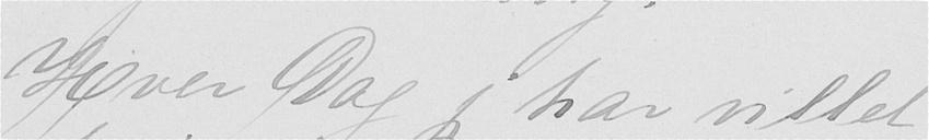Hver Dag jeg har villet
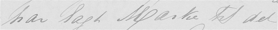har lagt Mærke til det
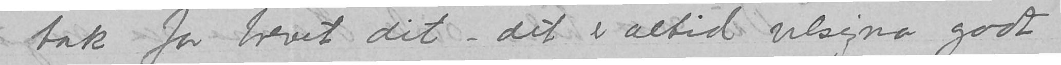tak for brevet dit – det er altid velsigna godt
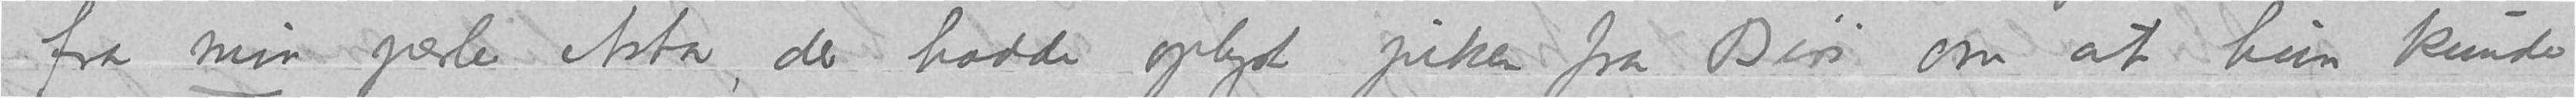fra min perle Asta, der hadde oplyst piken fra Biri om at hun kunde
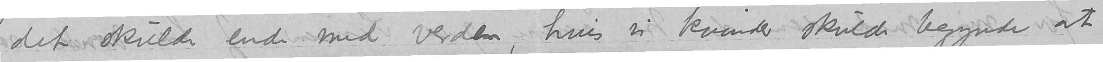det skulde ende med verden, hvis vi kvinder skulde begynde at
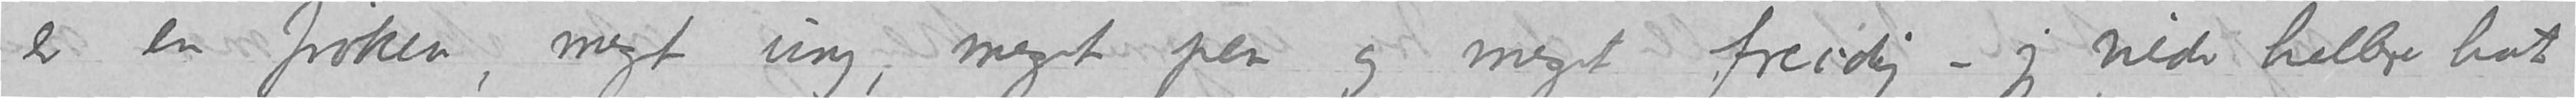er en frøken, meget ung, meget pen og meget freidig -  jeg vilde hellere hat
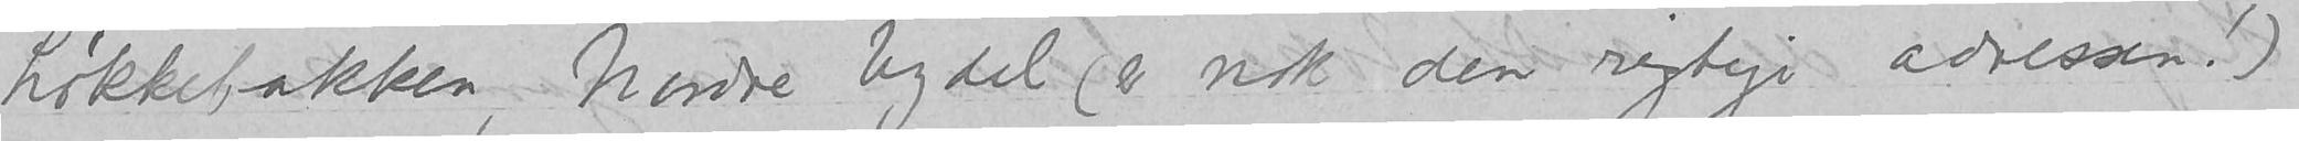Løkkebakken, Nordre bydel (er nok den rigtige adressen!)
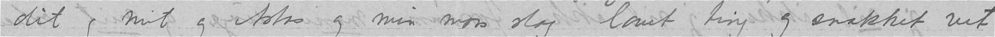dit og mit og Astas og min mors slag lavet ting og snakket vet –
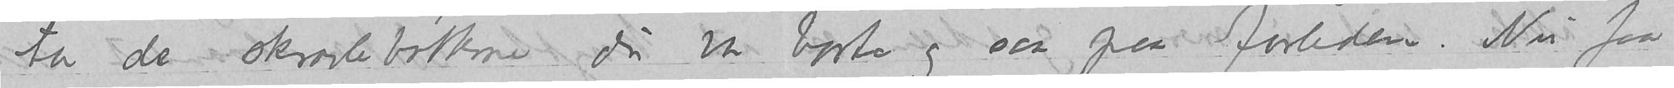ta de skravlebøtterne du var borte og saa paa forleden. Nu faar
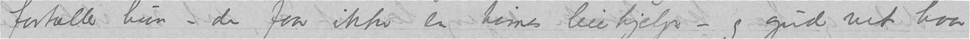fortæller hun – de faar ikke en times leiehjelp – og gud vet hva
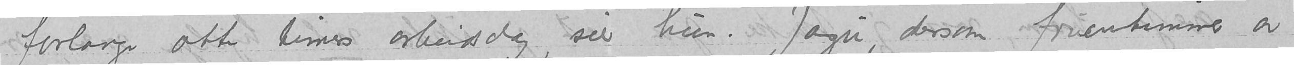forlange otte timers arbeidsdag, sier hun. Jagu, dersom fruentimmer av
